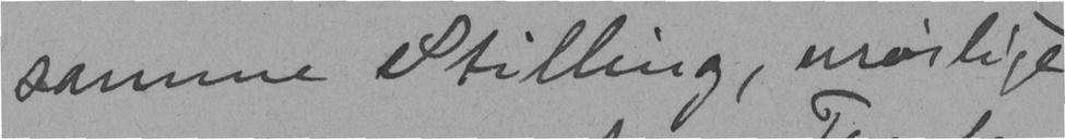samme Stilling, morlige
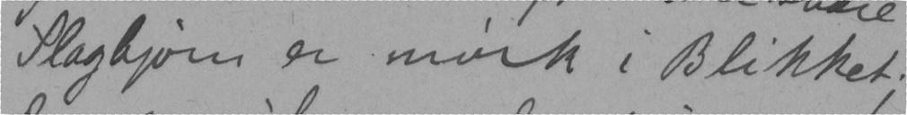Slagbjørn er mørk i Blikket;
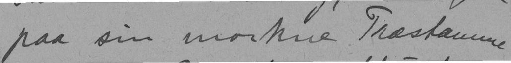paa sin morkne Træstamme
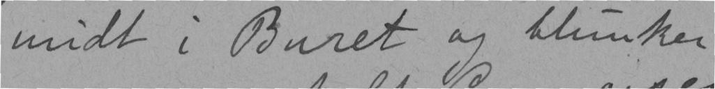midt i Buret og blunker
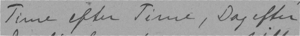Time efter Time, Dag efter
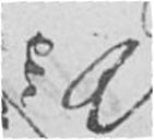en
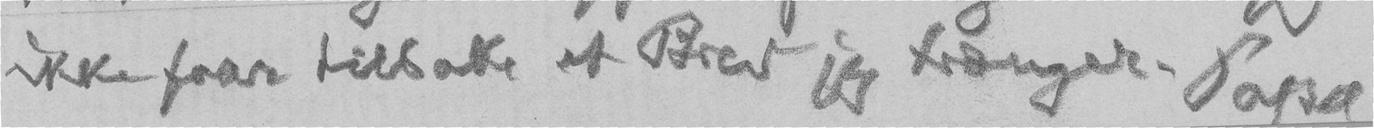ikke faar tilbake et Brev jeg trænger. Papa
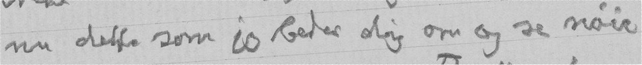nu dette som jeg beder dig om og se nøie
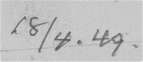18/4. 49.
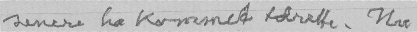senere ha kommet tilrette. Nu
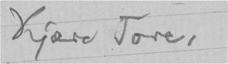Kjære Tore,
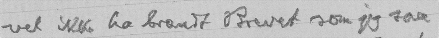vel ikke ha brændt Brevet som jeg saa
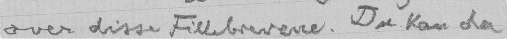over disse Fillebrevene. Du kan da
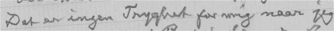Det er ingen Tryghet for mig naar jeg
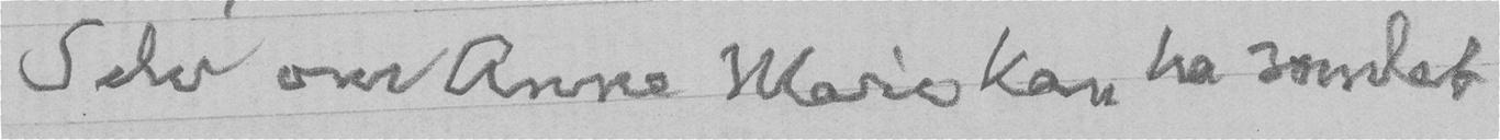Selv om Anne Marie kan ha somlet
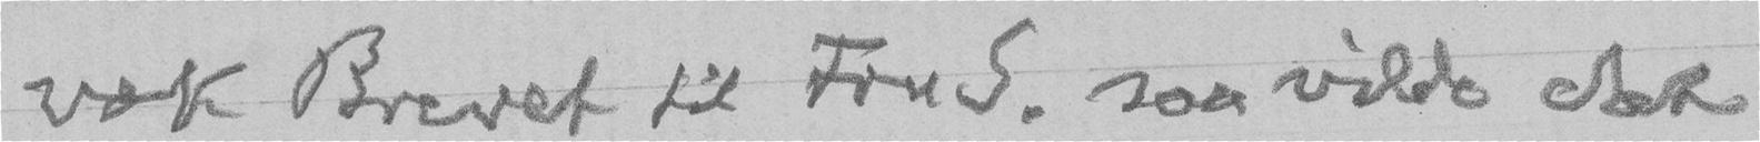væk Brevet til Fru S. saa vilde det
